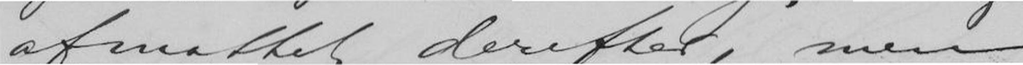afmattet derefter, men
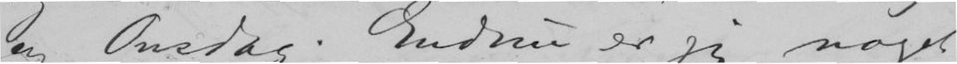og Onsdag. Endnu er jeg noget
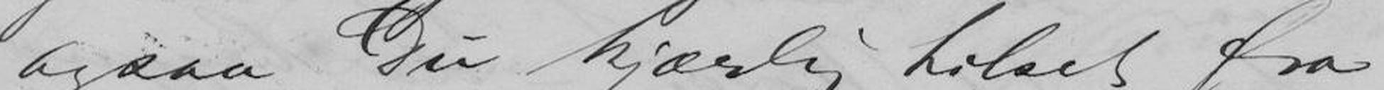ogsaa Du kjærlig hilset fra
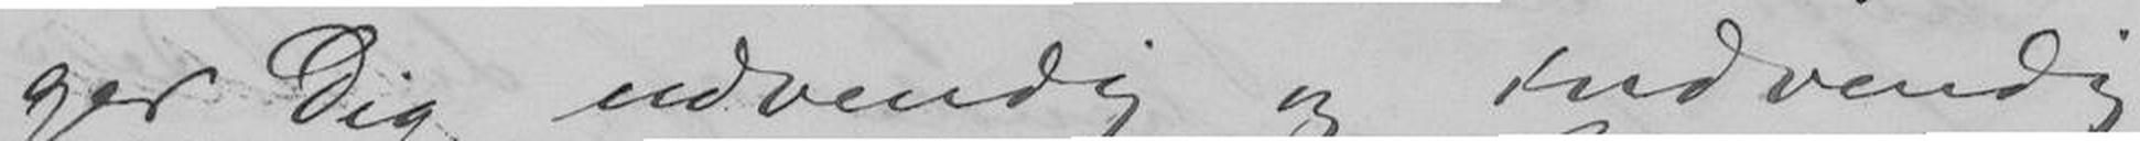ger Dig udvendig og indvendig
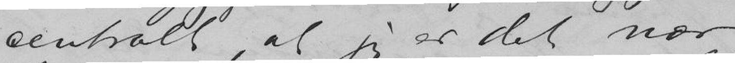centralt, at jeg er det nær,
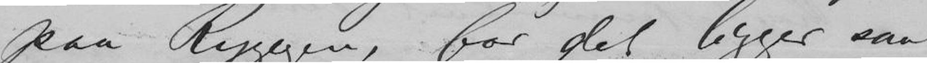paa Ryggen, for det ligger saa
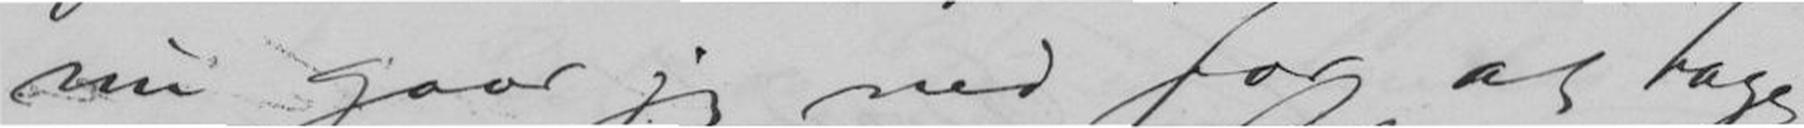nu gaar jeg ned for at tage
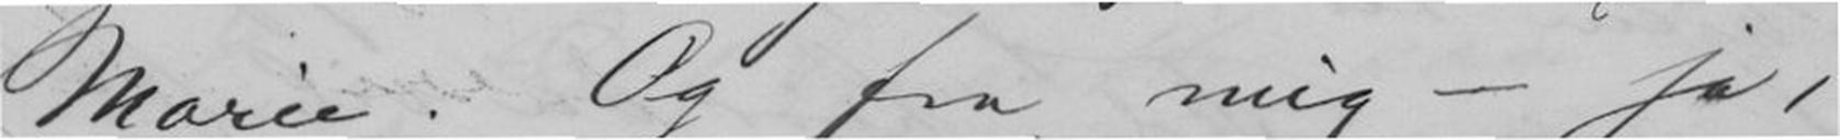Marie. Og fra mig - ja,
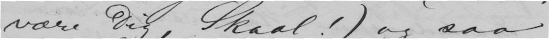være Dig, Skaal!) og saa
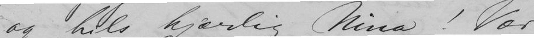og hils kjærlig Nina! Vær
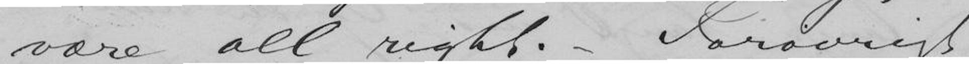være all right. - Forøvrigt
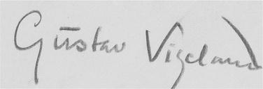Gustav Vigeland
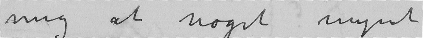mig at noget mynt
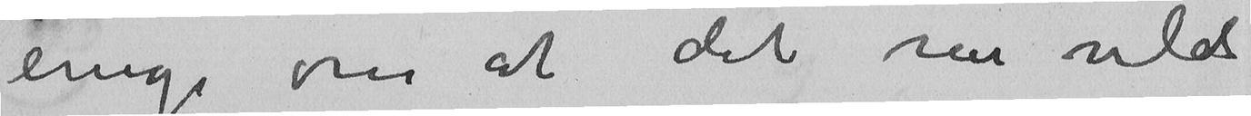enige om at det nu vilde
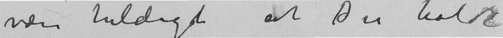være heldigt at Du holdt
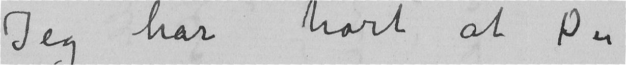Jeg har hørt at Du
